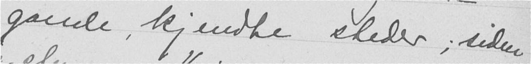gamle, kjendte steder; siden
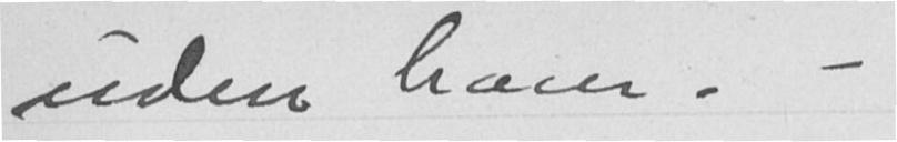uden ham. -
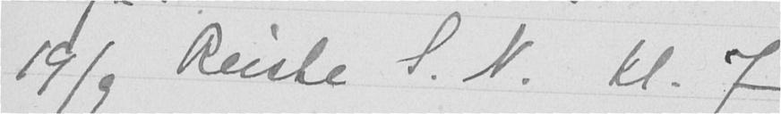19/9 Reiste S. N. kl. 7
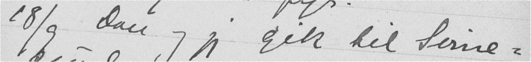18/9 Don og jeg gik til Svine-
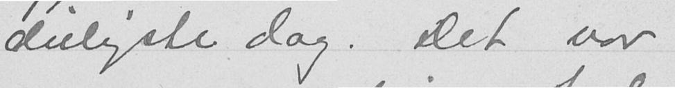deiligste dag. Det var
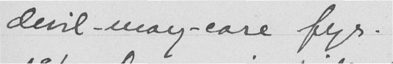devil-may-care fyr.
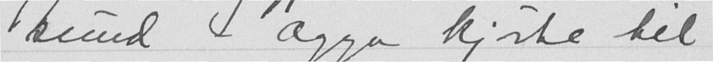sund - Agga kjørte til
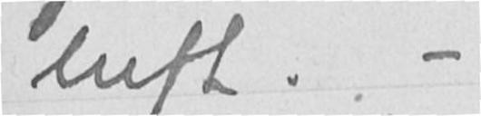luft. -
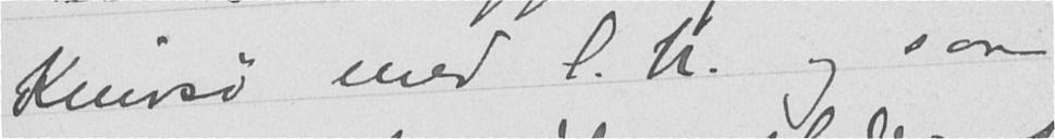Knivsø med S. N. og saa
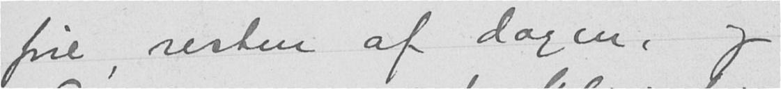frie, resten af dagen, og
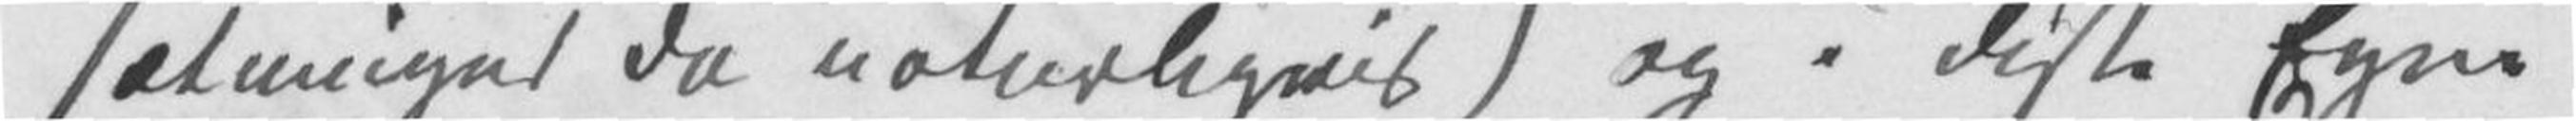sætninger da naturligvis) og i disse Egne
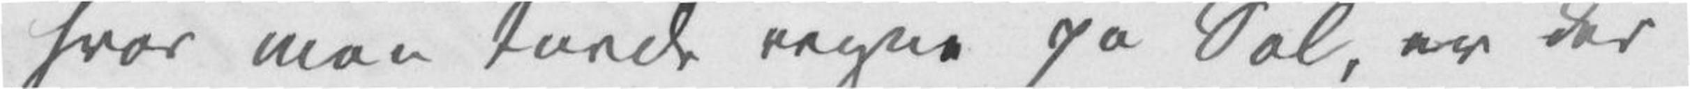hvor man turde regne på Sol, er der der
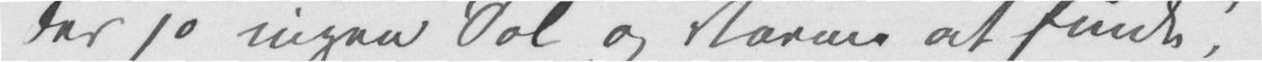der jo ingen Sol og Varme at finde!
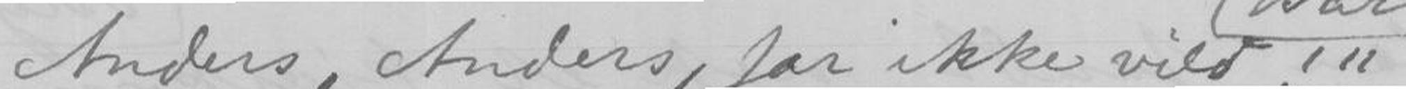Anders, Anders, far ikke vild!"
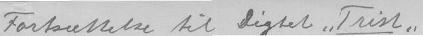Fortsættelse til Digtet "Trist"
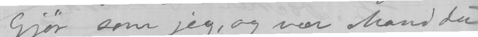Gjør som jeg, og vær Mand Du
